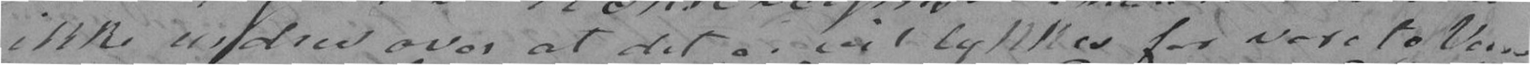ikke undres over at det ei vil lykkes for vore to Ven-
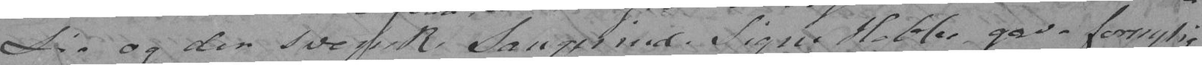Lie og den Svenske Sangerinde Signe Hebbe, gave fornylig
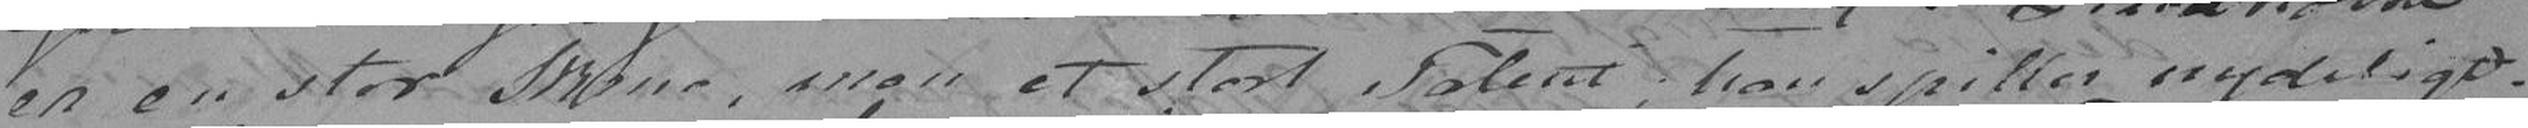er en stor Skrue, men et stort Talent; han spiller nydeligt.
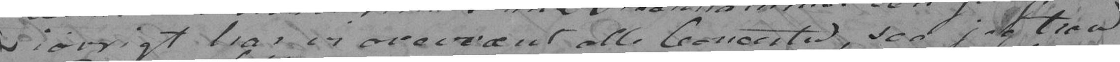iøvrigt har vi overværet alle Concerter, saa jeg troer
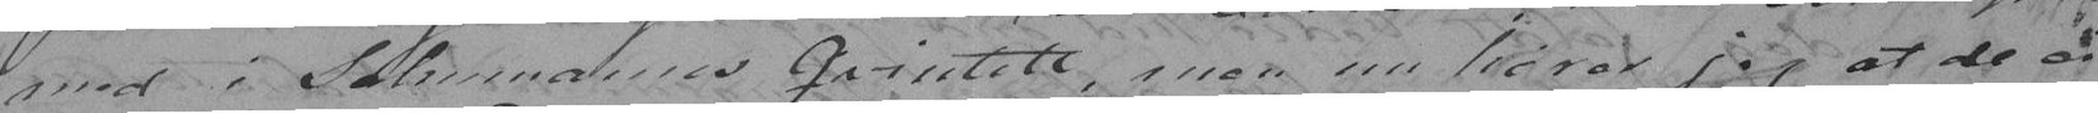med i Schumanns Qvintett, men nu hører jeg at de ei
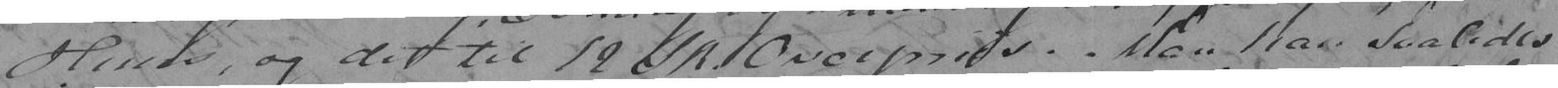Huus, og det til 12 Sk. Overpriis. Man kan saaledes
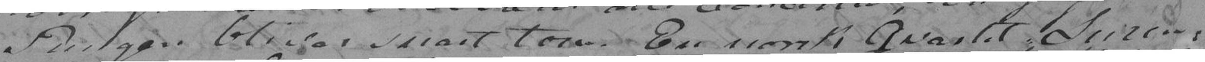Pungen bliver snart tom. En norsk Qvartet Luren,
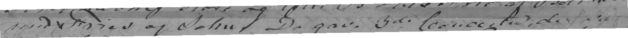med Fries og John. De gave 3de Concerten, der var
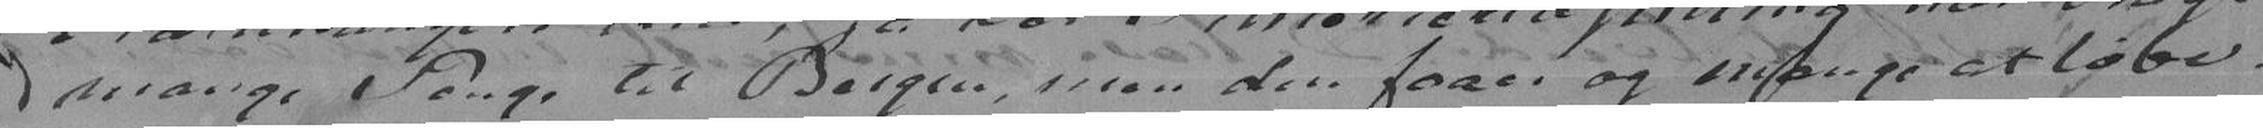mange Penge til Bergen, men den faaer og mange at løbe.
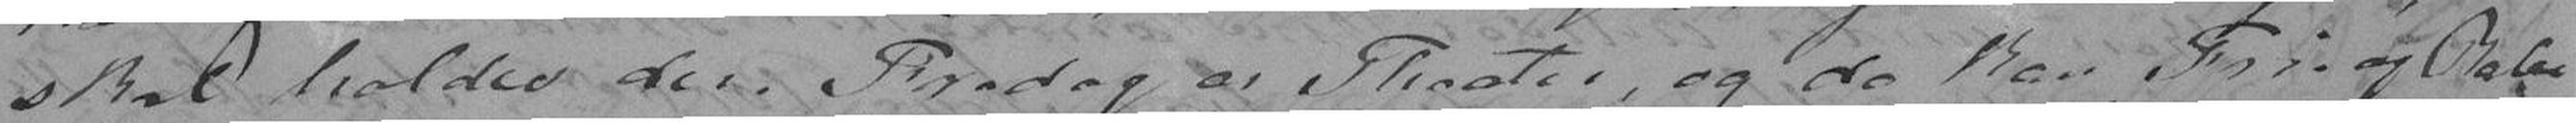skal holdes den Fredag er Theater, og da kan Fri. og Rabe
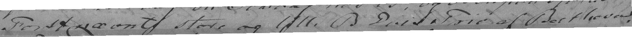Førstnæmte store og lille B Durs Trio af Beethoven,
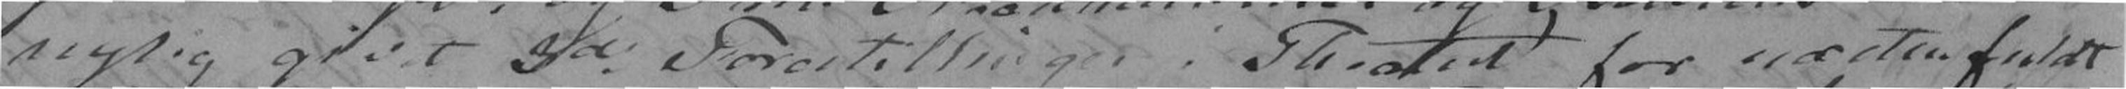nylig givet 3de Forestillinger i Theatret for næsten fuldt
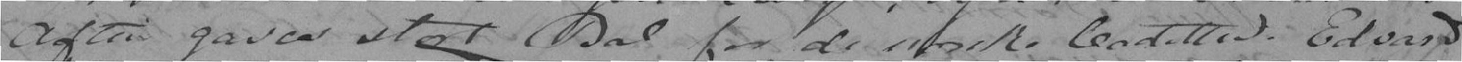Aften gaves stort Bal for de norske Cadetter. Edvard
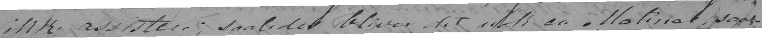ikke assistere; saaledes bliver det nok en Matine saa-
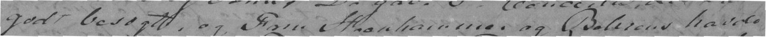godt besøgt, og Frue Steenhammer og Behrens havde
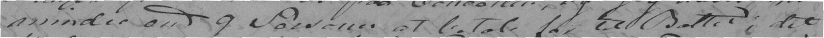mindre end 9 Personer at betale for til Ballet; det
